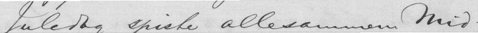Juledag spiste allesammen Mid-
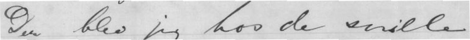Der blev jeg hos de snille
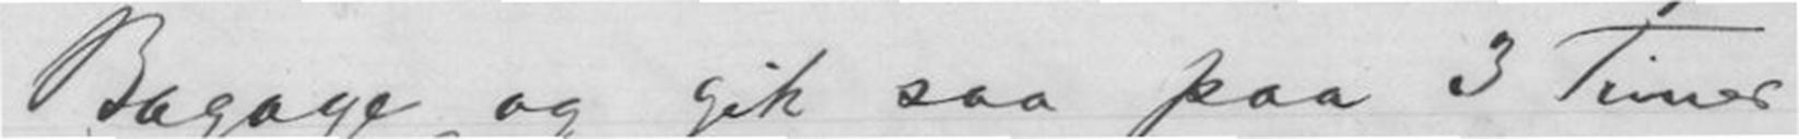Bagage og gik saa paa 3 Timer
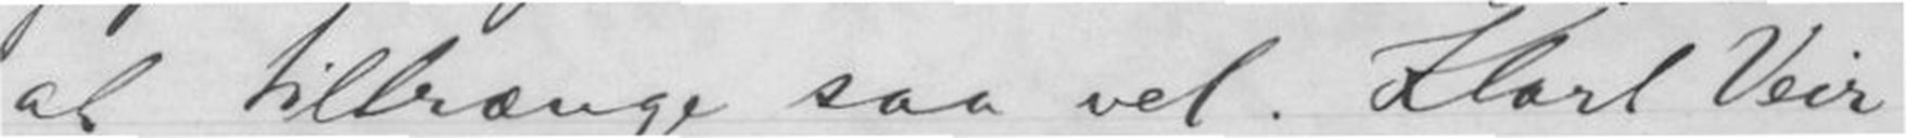at tiltrænge saa vel. Klart Veir
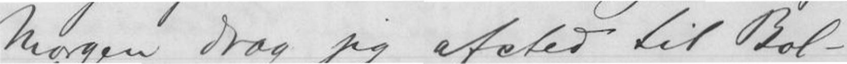Morgen drog jeg afsted til Bol-
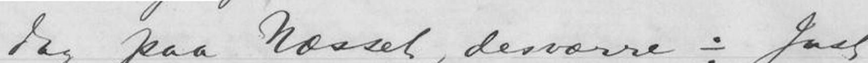dag paa Næsset, desværre -minus Just
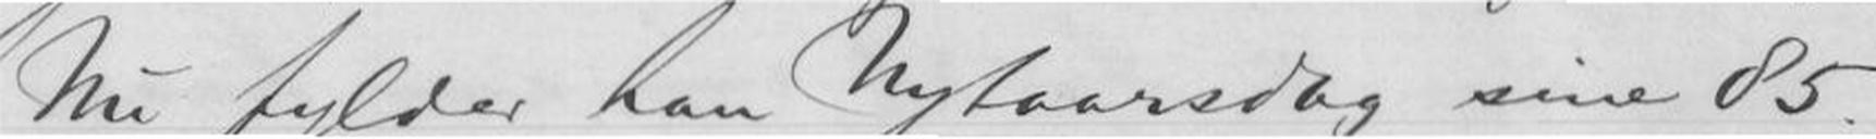Nu fylder han Nytaarsdag sine 85.
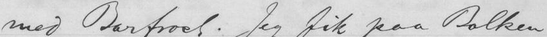med Barfrost. Jeg fik paa Bolken
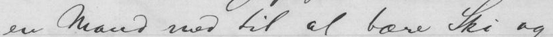en Mand med til at bære Ski og
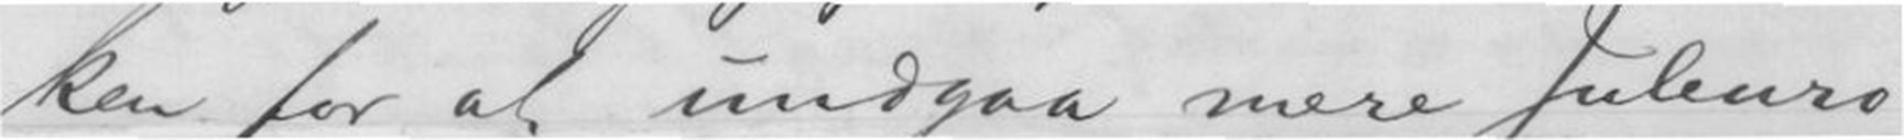ken for at undgaa mere Juleuro
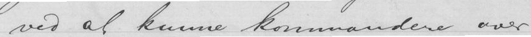ved at kunne kommandere over
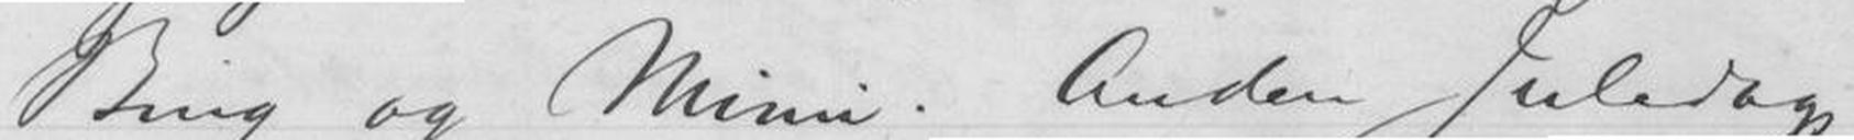Bing og Mimi. Anden Juledag
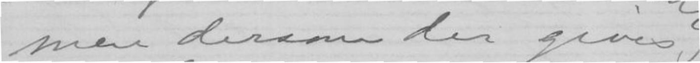men dersom der gives
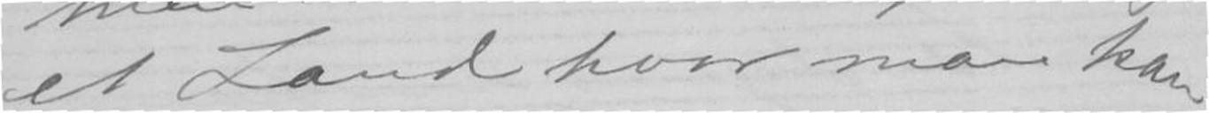et Land hvor man kan
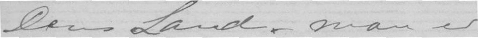Deres Land, man er
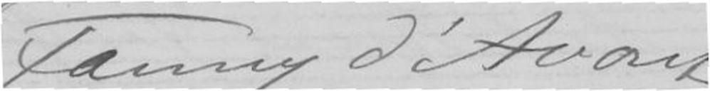Fanny d'Avont
